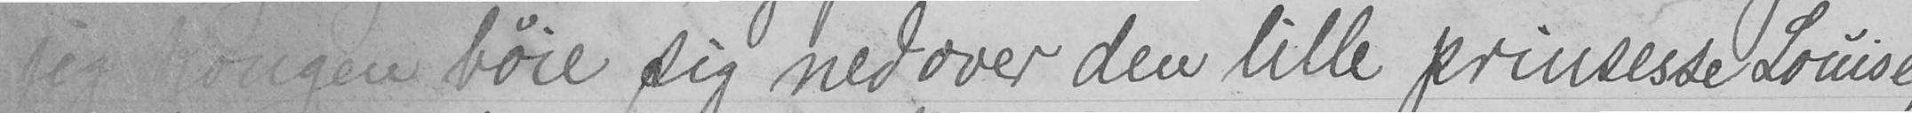jeg kongen bøie sig nedover den lille prinsesse Louise,
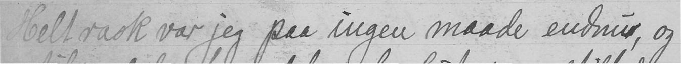Helt rask var jeg paa ingen maade endnu, og
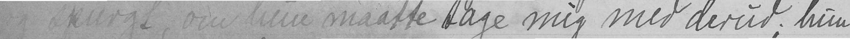og spurgt, om hun maatte tage mig med derud. hun
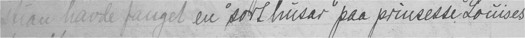stian havde fanget en "sort husar" paa prinsesse Louises
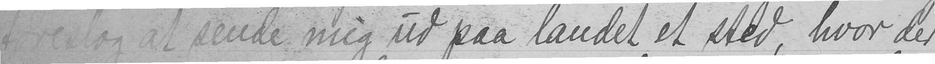foreslog at sende mig ud paa landet et sted hvor der
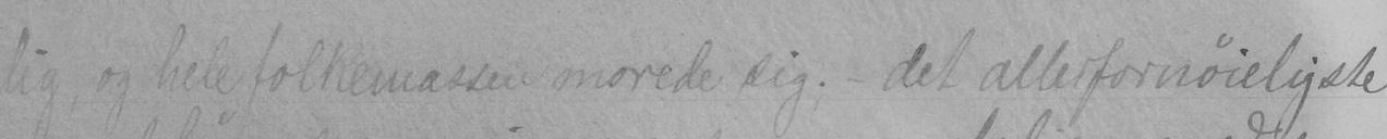lig, og hele folkemassen morede sig; - det allerfornoieligste
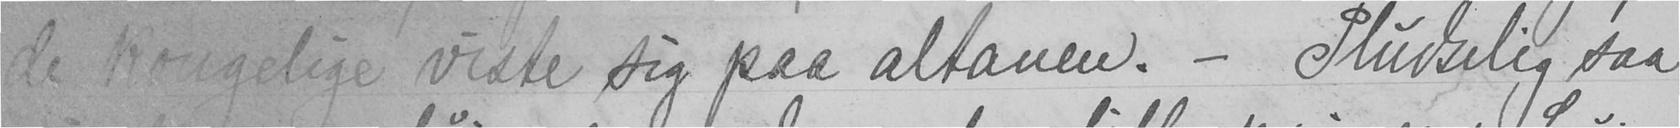de kongelige viste sig paa altanen. - Pludselig saa
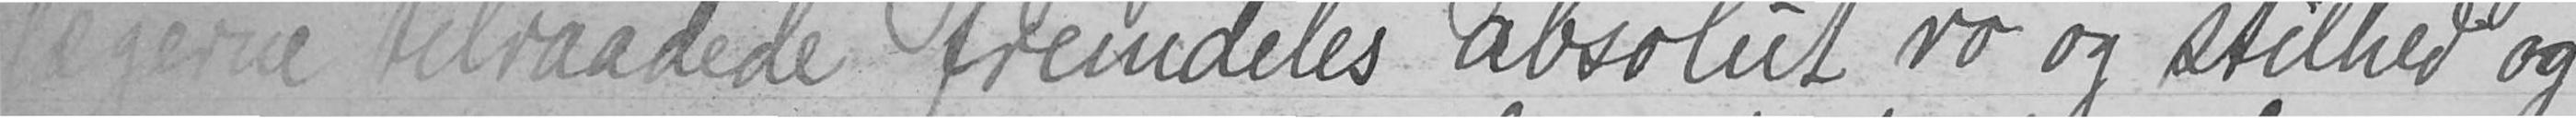legerne tilraadede fremdeles absolut ro og stilhed og
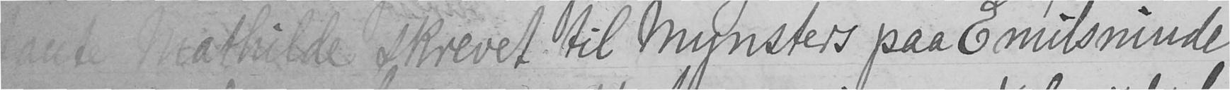tante Mathilde skrevet til Mynsters paa Emilsminde
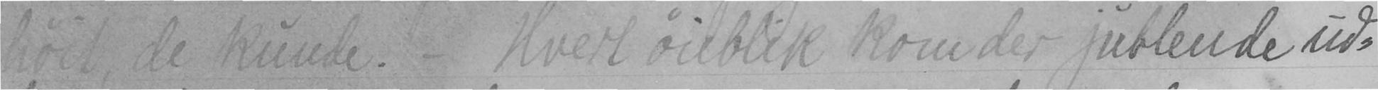høit, de kunde. - Hvert øieblik kom der jublende ud-
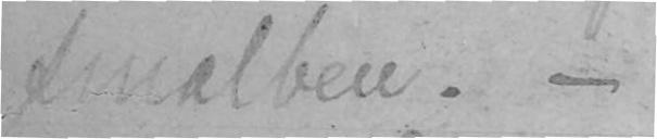smalben. -
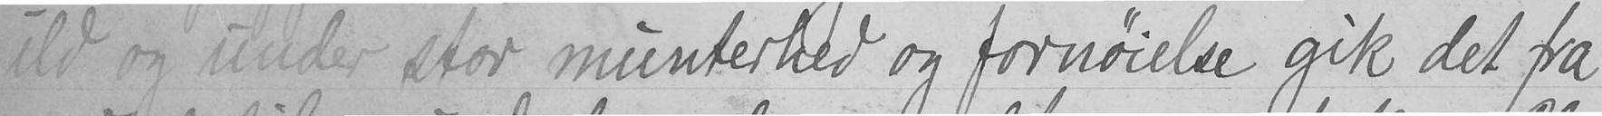ild og under stor munterhed og fornøielse gik det fra
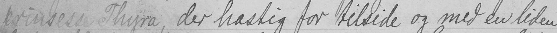prinsesse Thyra, der hastig for tilside og med en liden
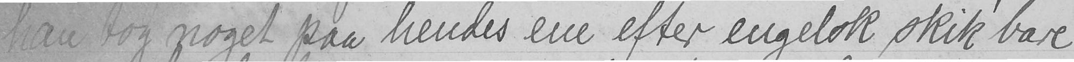han tog noget paa hendes ene efter engelsk skik bare
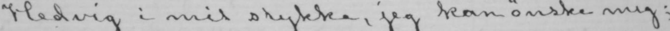Hedvig i mit stykke, jeg kan ønske mig;
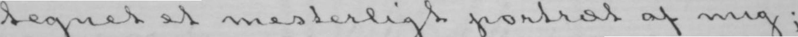tegnet et mesterligt portræt af mig;
